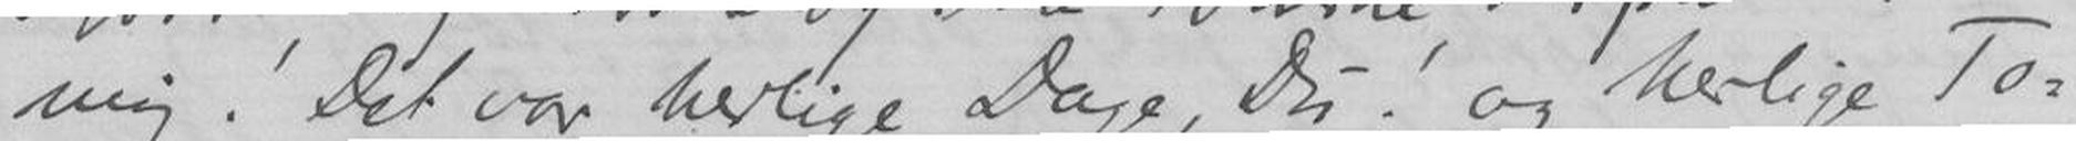mig! Det var herlige Dage, Du! og herlige To-
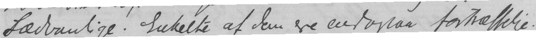Sædvanlige. Enkelte af den er endogsaa fortræffelige.
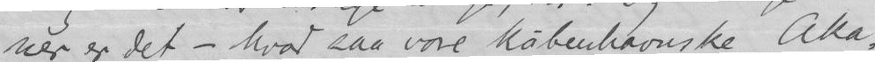ner er det - hvad saa vore københavnske Aka-
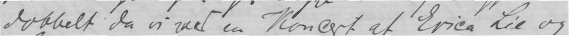dobbelt da vi ved en Koncert af Erica Lie og
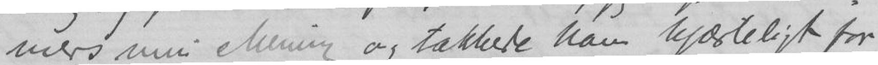mers min Mening og takkede han hjærteligt for
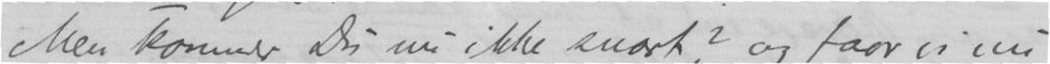Men kommer Du nu ikke snart? og faar vi nu
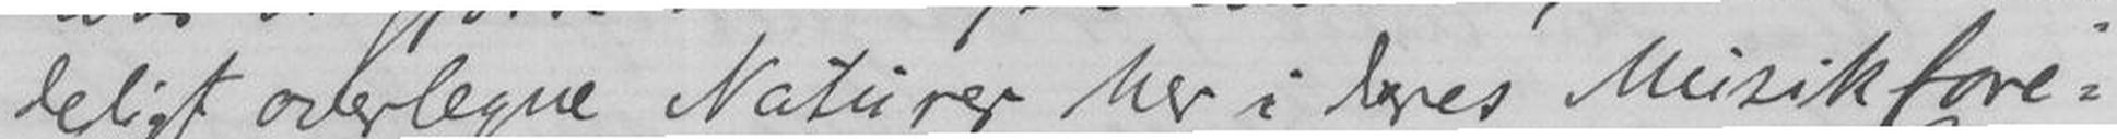deligt overlegne Naturer her i deres Musikfore-
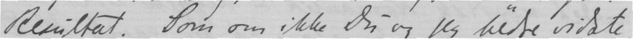Resultat. Som om ikke Du og jeg bedre vidste,
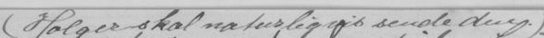(Holger skal naturligvis sende den)
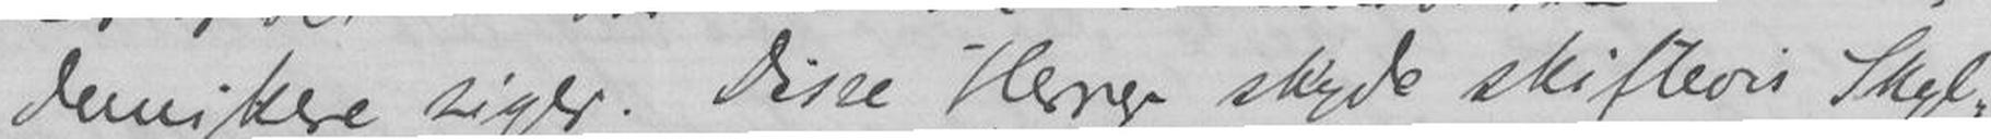demiske siger. Disse Herrer skyde skiftevis Skyl-
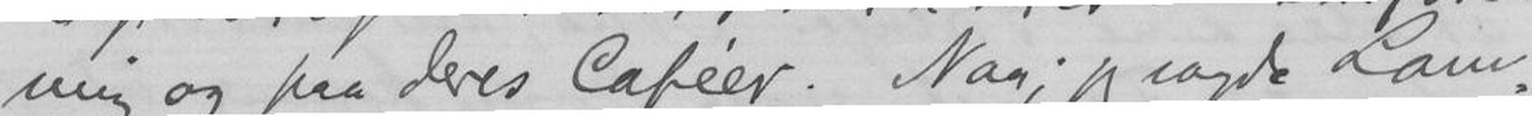ning og paa deres Caféer. Naa, jeg sagde Lam-
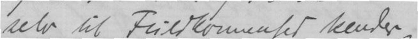selv til Fuldkommenhed hender
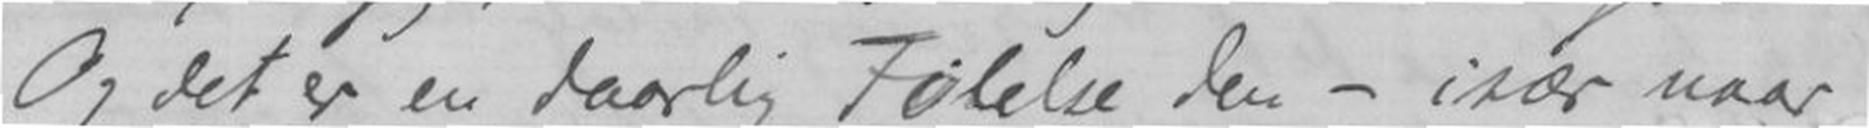Og det er en daarlig Følelse den - især naar
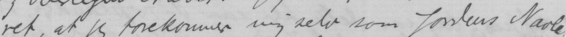ret, at jeg forekommer mig selv som Jordens Navle.
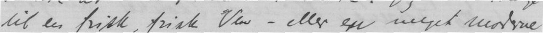til en frisk, frisk Ven - eller en meget moderne
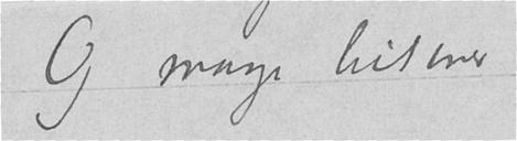Og mange hilsener
